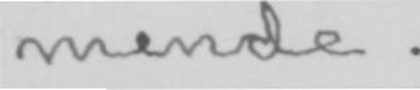mende.
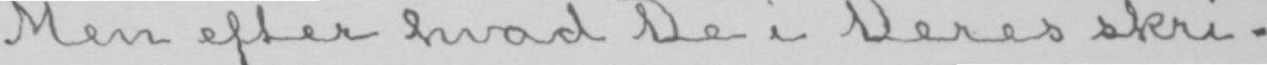Men efter hvad De i Deres skri-
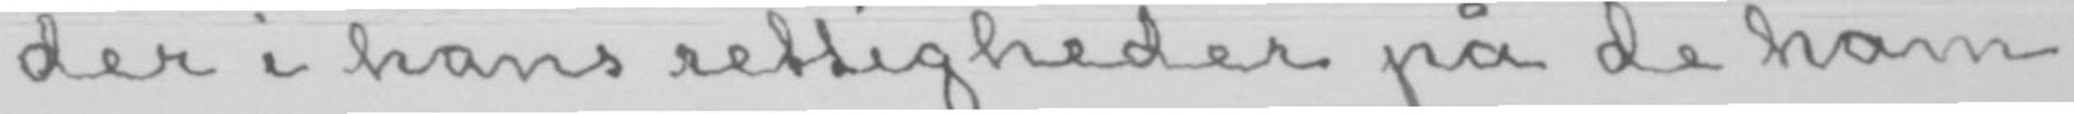der i hans rettigheder på de ham
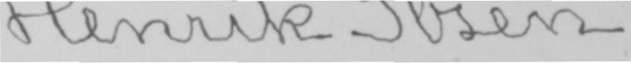Henrik Ibsen.
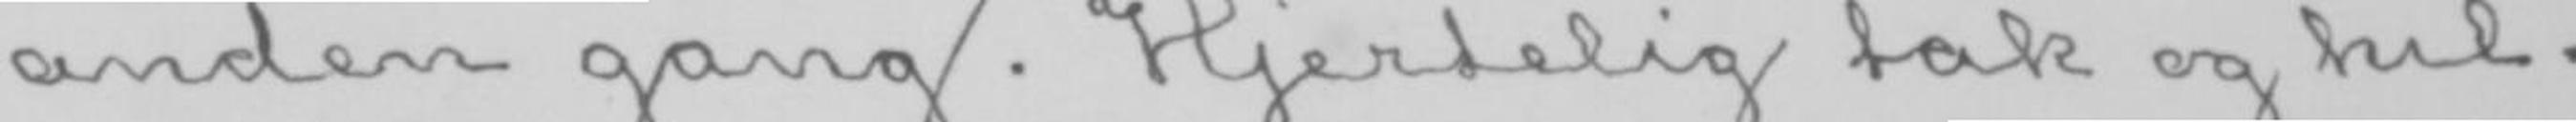anden gang. Hjertelig tak og hil-
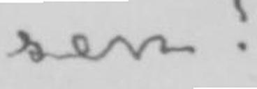sen!
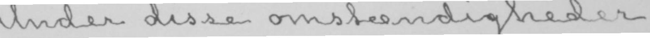Under disse omstændigheder
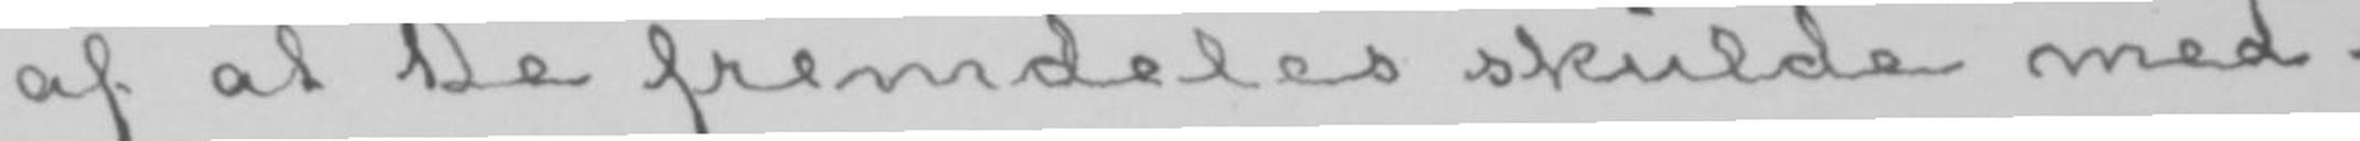af at De fremdeles skulde med-
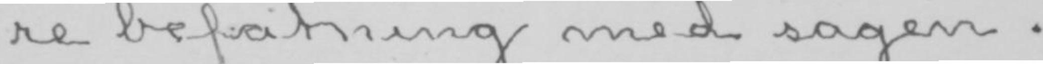re befatning med sagen.
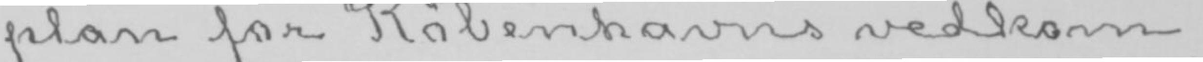plan for Københavns vedkom-
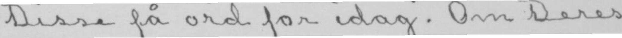Disse få ord for idag. Om Deres
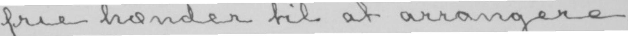frie hænder til at arrangere
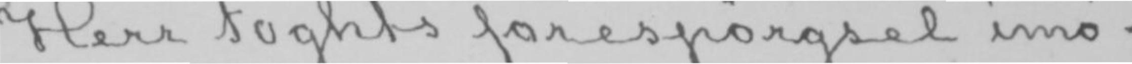Herr Foghts forespørgsel imø-
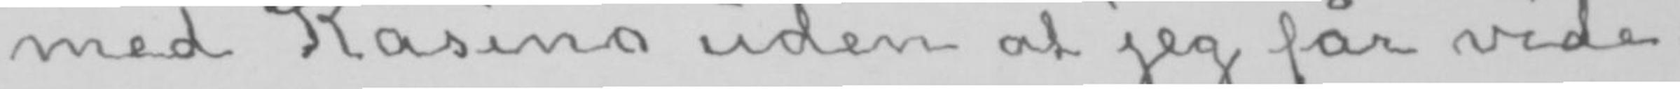med Kasino uden at jeg får vide-
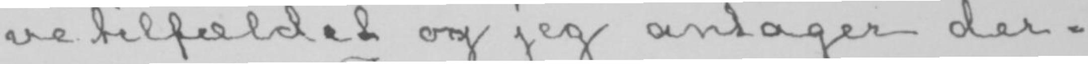ve tilfældet og jeg antager der-
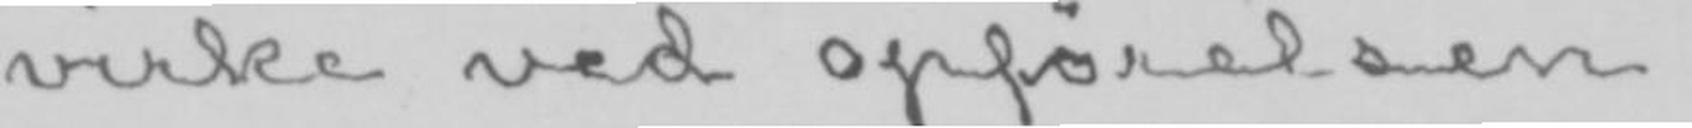virke ved opførelsen.
